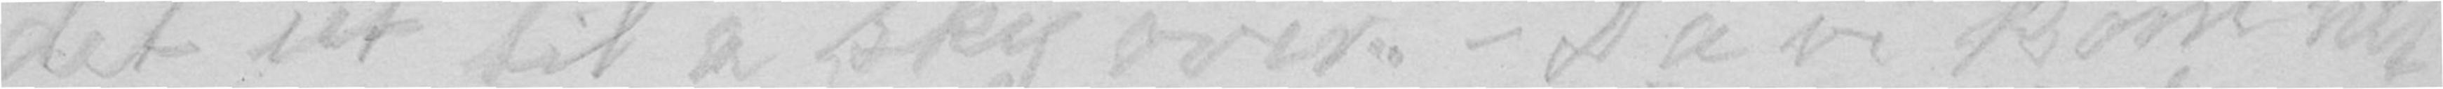det ut til å sky over. - Da vi kom hit
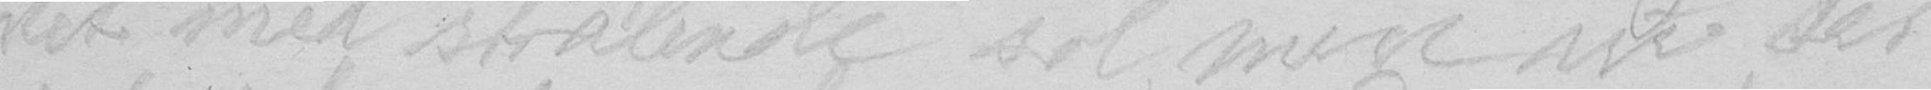det med strålende sol, men nu ser
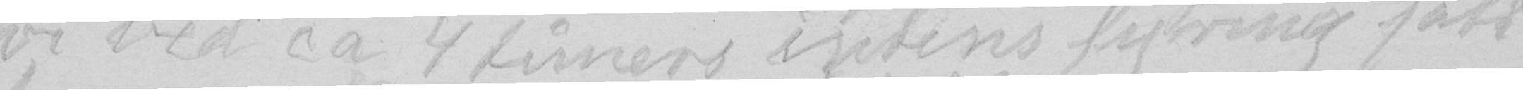vi ved ca 4 timers intens fyring fått
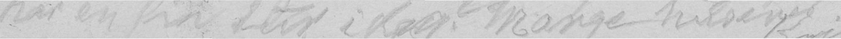har en fin tur i dag? Mange hilsener
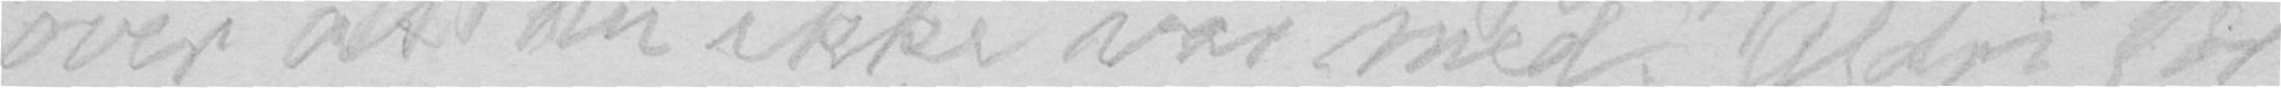over at du ikke var med, aldri før
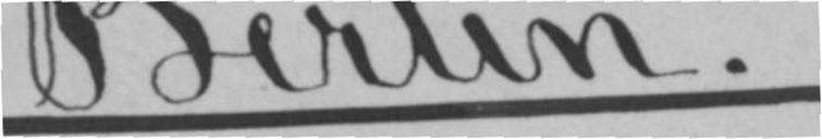Berlin.
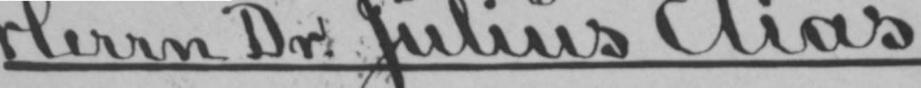Herrn Dr. Julius Elias,
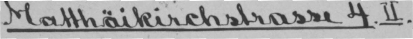Matthäikirchstrasse 4. II.
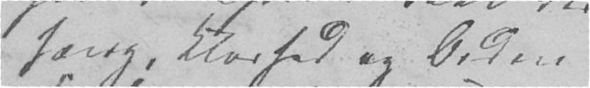hæng, Klarhed og Orden
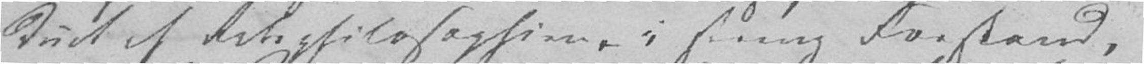duct af Retsphilosophien i streng Forstand,
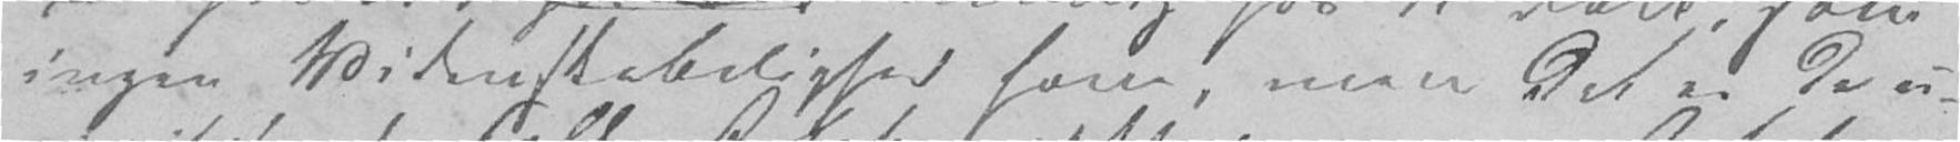ingen Videnskabelighed have, men det er de u-
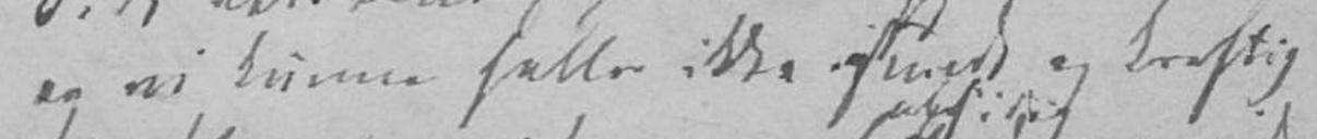og vi kunne heller ikke finde og kraftig
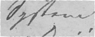System
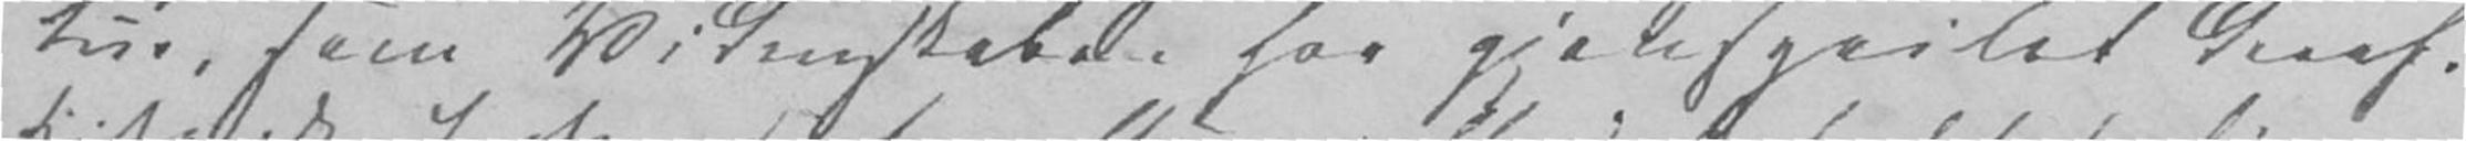tur, som Videnskaben har gjenspeilet deraf.
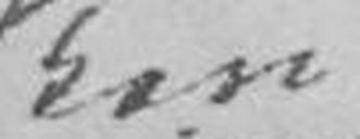kan
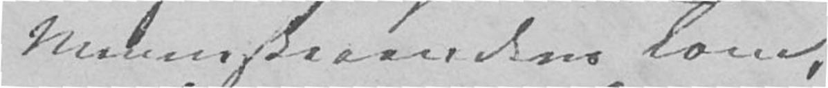Menneskehedens Love,
